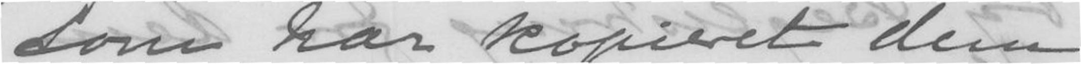som har kopiert dem
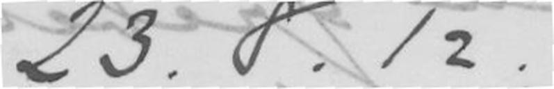23.8.12
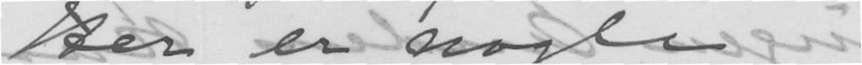Her er nogle
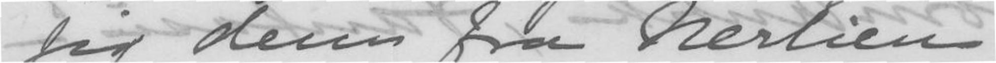jeg dem fra Herlien,
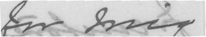for mig!
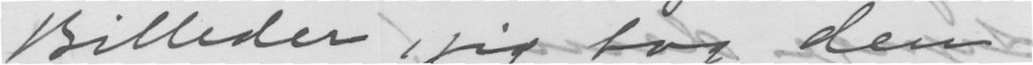Billeder, jeg tog den
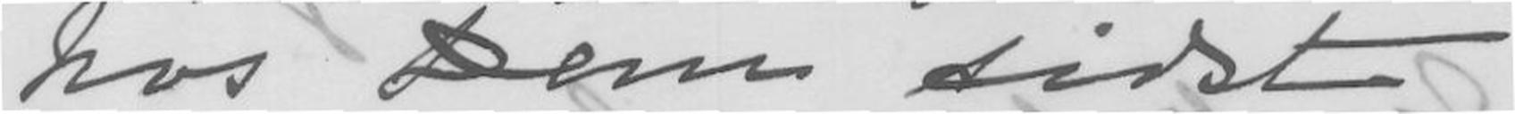hos Dem Sist!
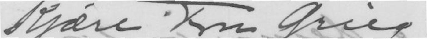Kjære Fam. Grieg!
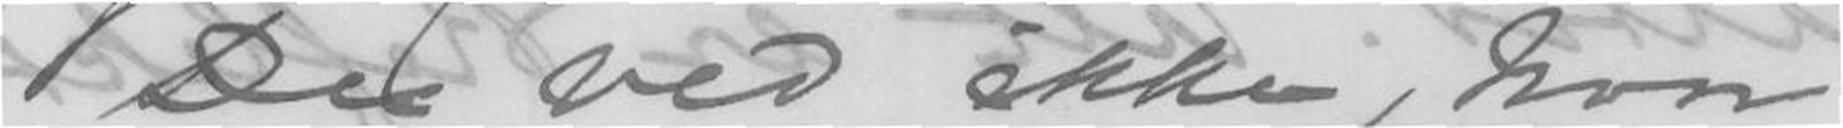De ved ikke, hvor
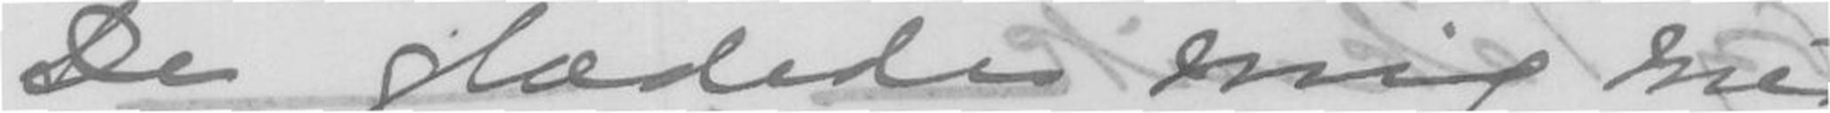De glædede mig med
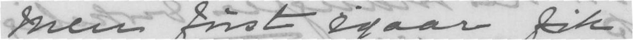men først igaar fik
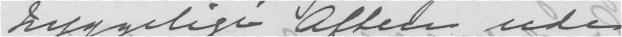hyggelige Aften ude
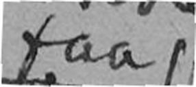faa
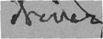driver
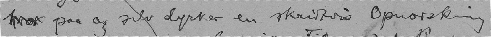tror paa og selv dyrker en skridtvis Opnorsking
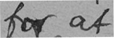for at
